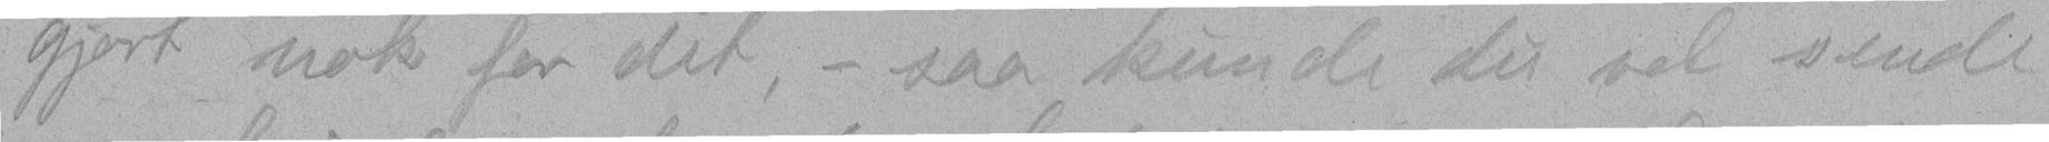gjort nok for det, - saa kunde du vel sende
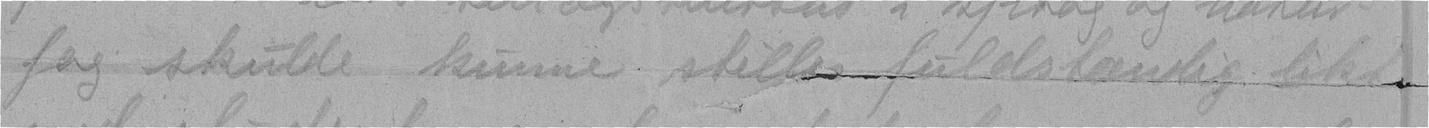fag skulde kunne stille fuldstændig likt
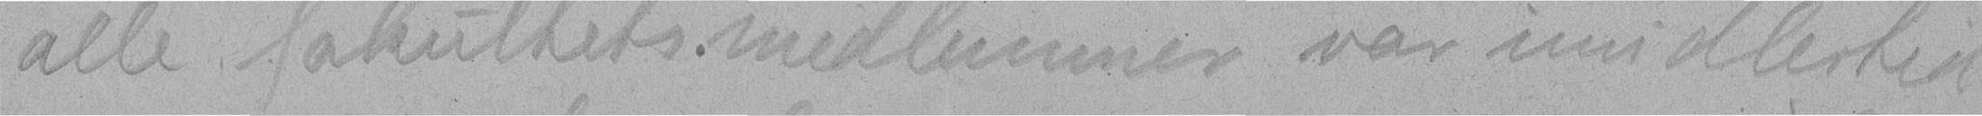alle fakultetsmedlemmer var imidlertid
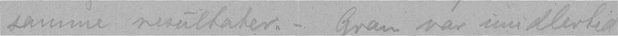samme resultater. - Gran var imidlertid
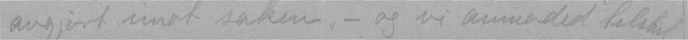avgjort imot saken, - og vi anmoded tilslut
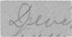Devik
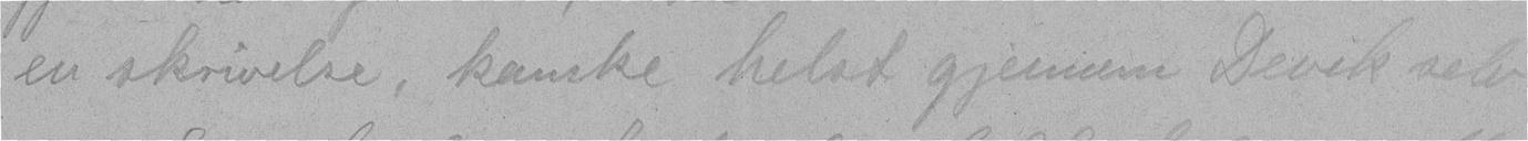en skrivelse, kanske helst gjennem Devik selv.
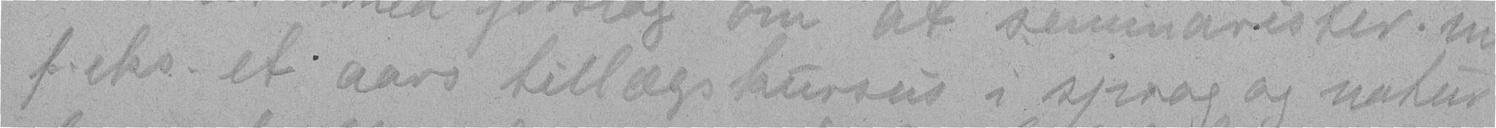f.eks. et aars tillægskursus i sprog og natur-
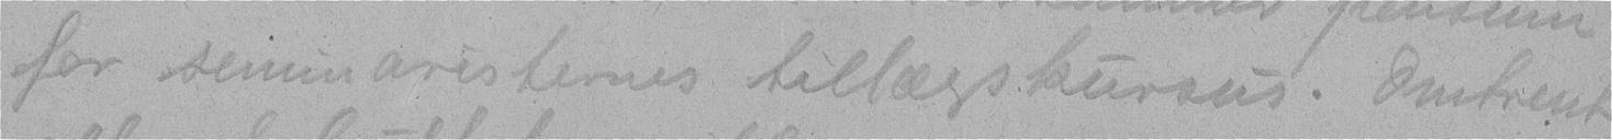for seminaristernes tillægskursus. Omtrent
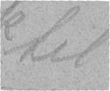til
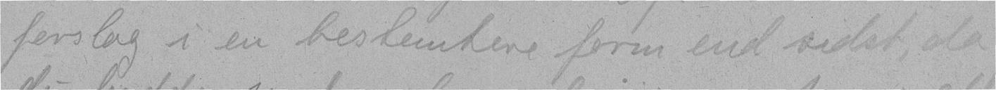forslag i en bestemtere form end sidst, da
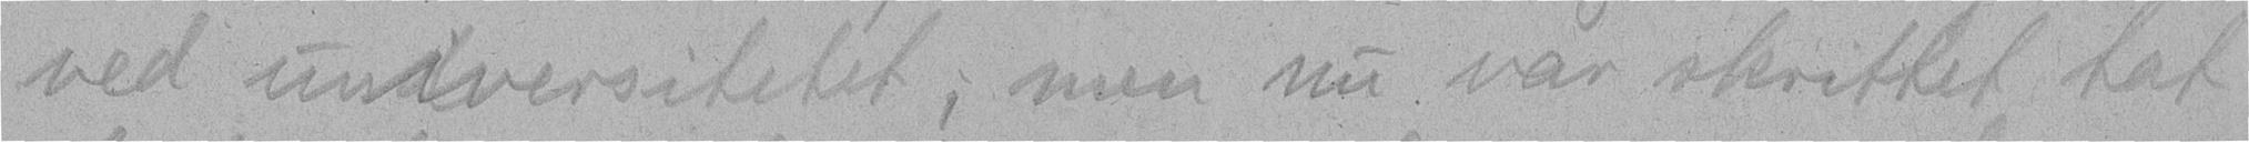ved unversitetet; men nu var skrittet tat
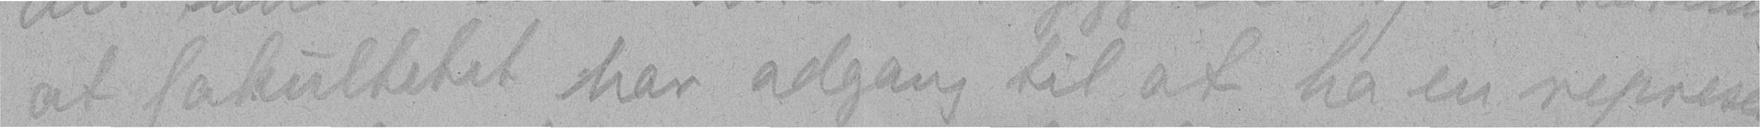at fakultetet har adgang til at ha en represen-
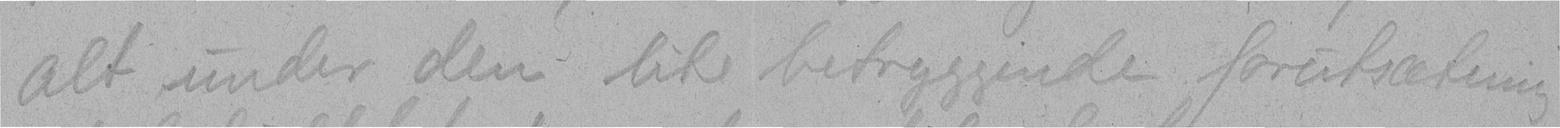alt under den lite betryggende forutsætning
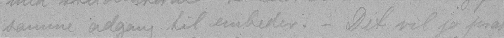samme adgang til embeder. - Det vil jo prak-
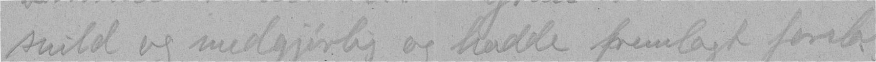snild og medgjørlig og hadde fremlagt forslag
